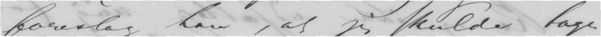foreslog han, at jeg skulde tage
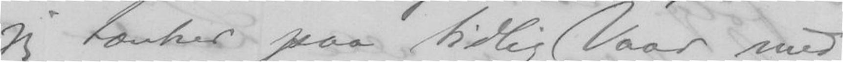jeg tænker paa tidlig Vaar med
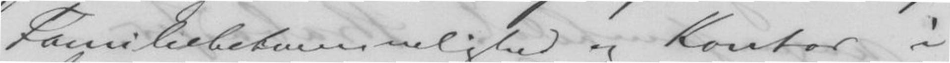Familiebekvemmelighed og Kontor i
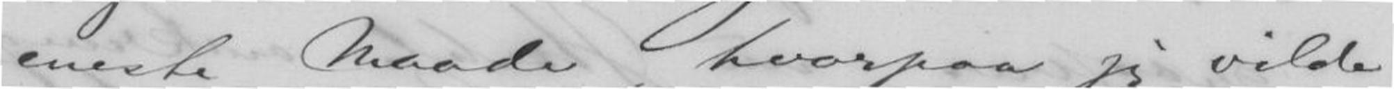eneste Maade, hvorpaa jeg vilde
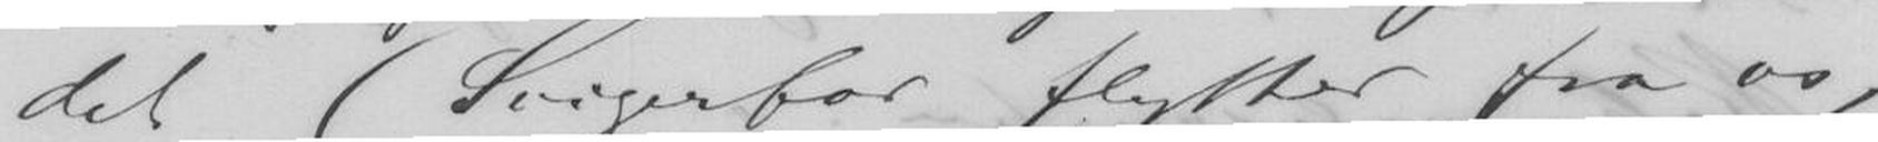det (Svigerfar flytter fra os,
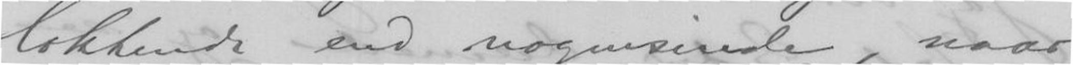lokkende end nogensinde, naar
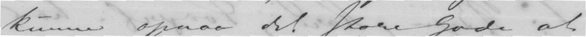kunne opnaa det store Gode at
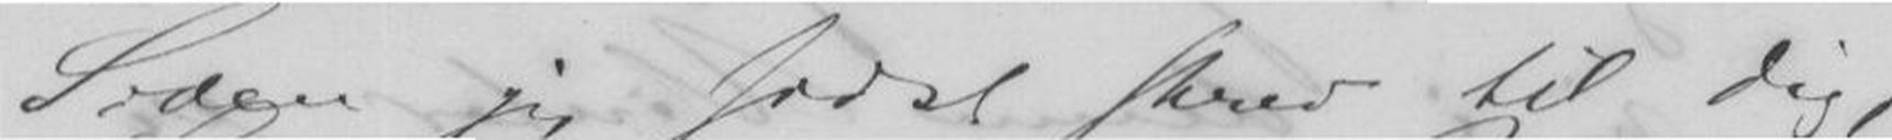Siden jeg sidst skrev til dig,
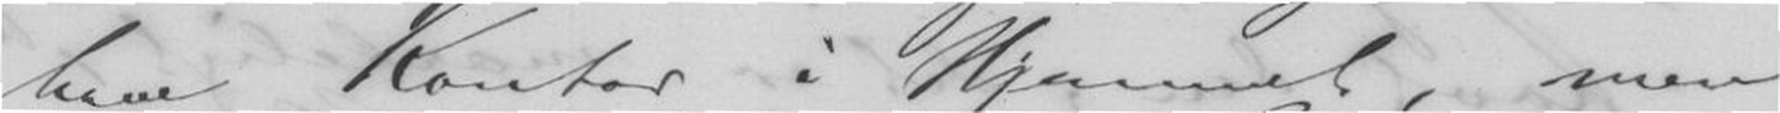have Kontor i Hjemmet, men
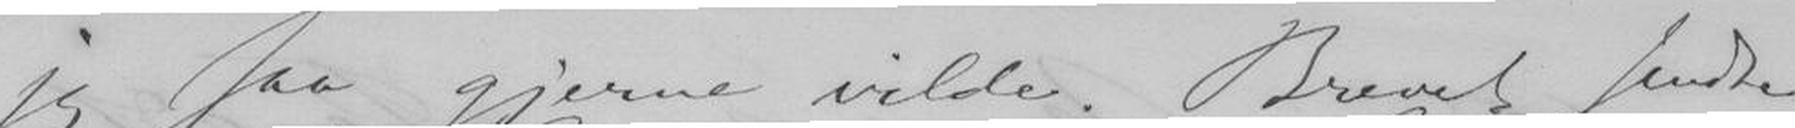jeg saa gjerne vilde. Brevet sendte
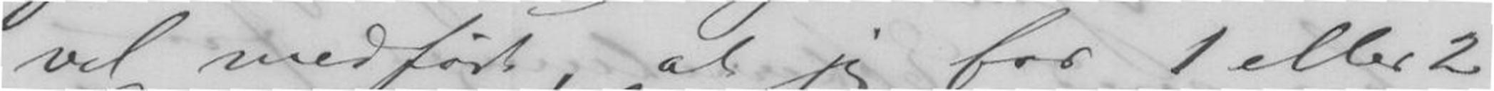vel medført, at jeg for 1 eller 2
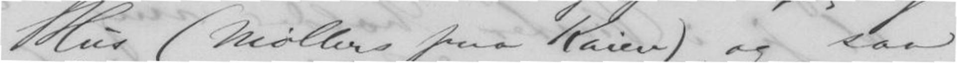Hus (Møllers paa Kaien) og saa
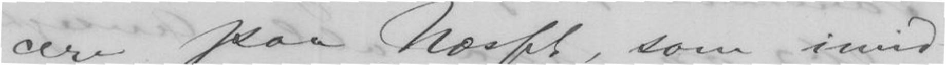cere paa Næsset, som imid-
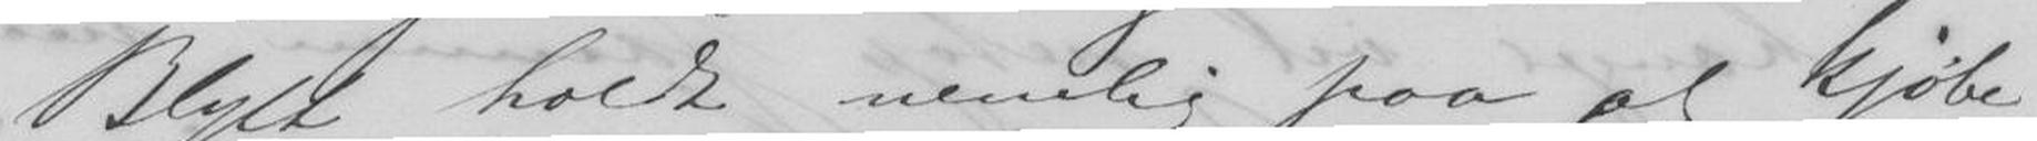Blytt holdt nemlig paa at kjøbe
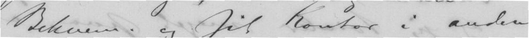Bekvem og sit Kontor i anden
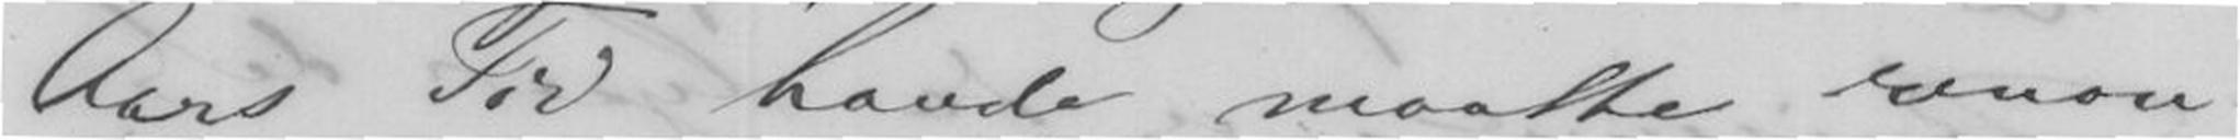Aars Tid havde maatte renon-
